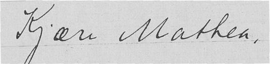Kjære Mathea,
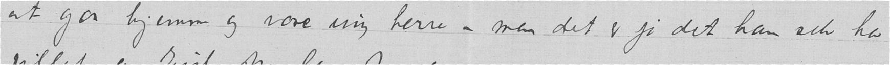at gaa hjemme og være ung herre – men det er jo det han selv har
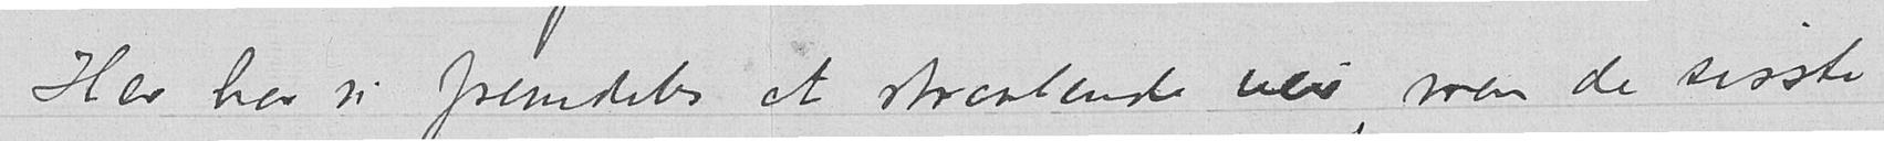Her har vi fremdeles et straalende veir, men de sisste
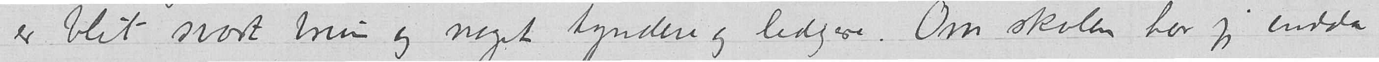er blit svært brun og noget tyndere og ledigere. Om skolen har jeg endda
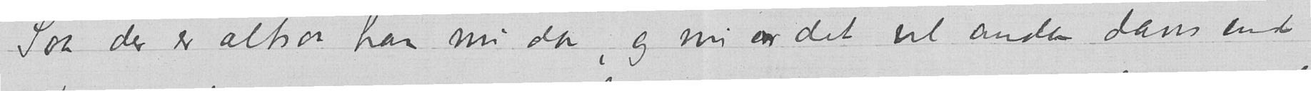Saa der er altsaa han nu da, og nu er det vel anden dans end
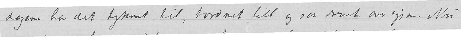dagene har det tyknet til, tordnet litt og saa drevet over igjen. Nu
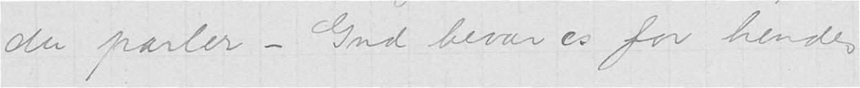du parler - Gud bevares for hendes
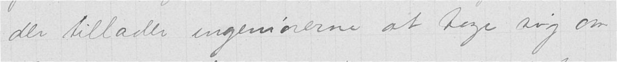der tillader ingeniørerne at tage sig om
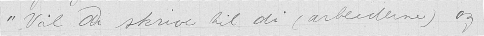"Vil De skrive til di (arbeiderne) og
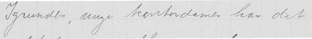Igrunden, unge kontordamer har det
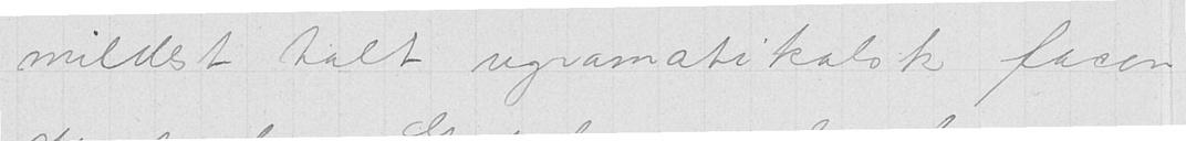mildest talt ugramatikalsk facon
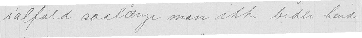ialfald saalænge man ikke beder hende
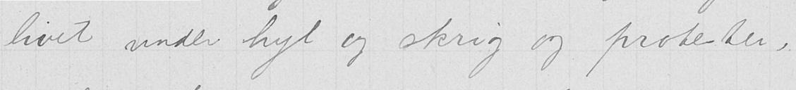livet under hyl og skrig og protester,
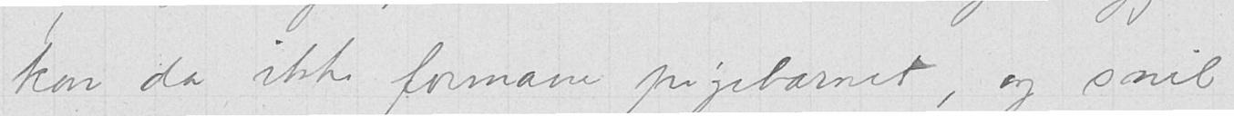kan da ikke formane pigebarnet, og snil
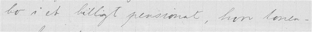bo i et billigt pensionat, hvor tonen -
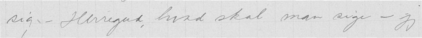sig. - Herregud, hvad skal man sige - jeg
